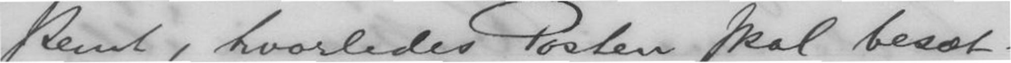stemt, hvorledes Posten skal besæt-
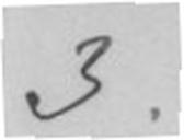3.
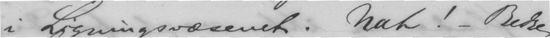i Ligningsvæsenet. Nah! - Bedre
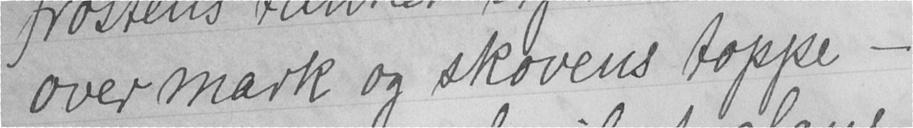over mark og skovens toppe -
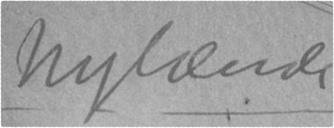Nylænde
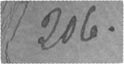206.
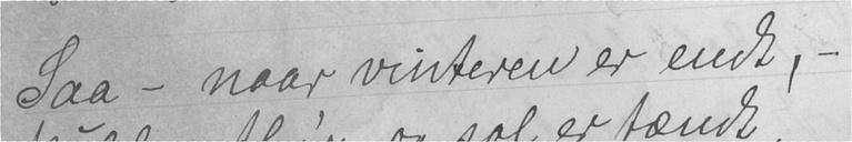Saa - naar vinteren er endt, -
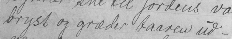bryst og græder taaren ud -
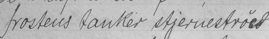frostens tanker stjernestrøed
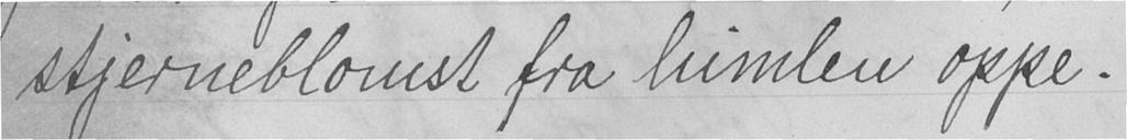stjerneblomst fra himlen oppe.
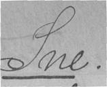Sne.
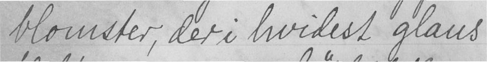blomster, der i hvidest glans
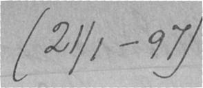(21/1-97)
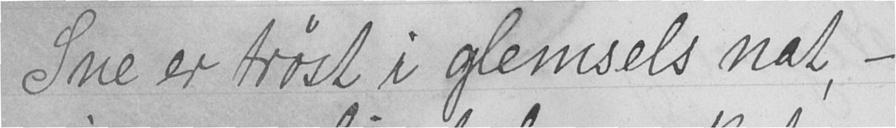Sne er trøst i glemsels nat, -
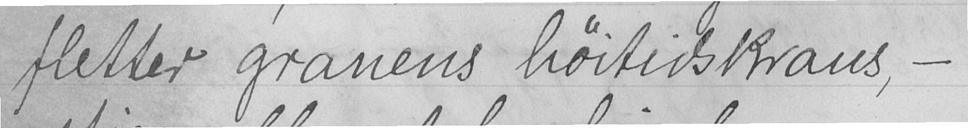fletter granens høitidskrans, -
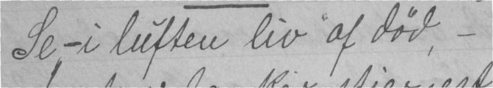Se - i luften liv af død, -
